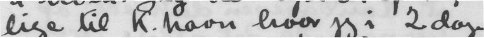lige til K.havn hvor jeg i 2 dager
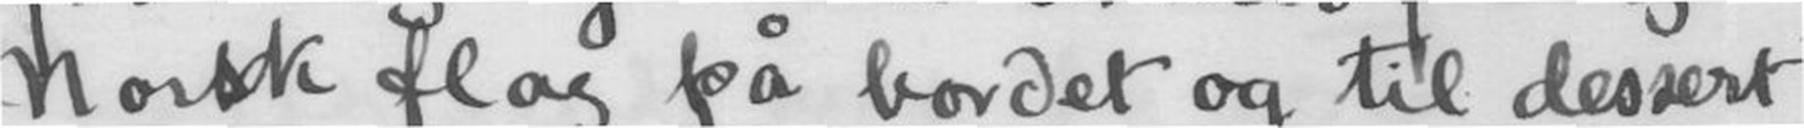Norsk flag på bordet og til dessert
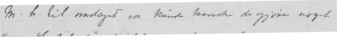M.h. til omslaget saa kunde kanske der gjøres noget
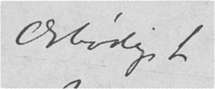Ærbødigst
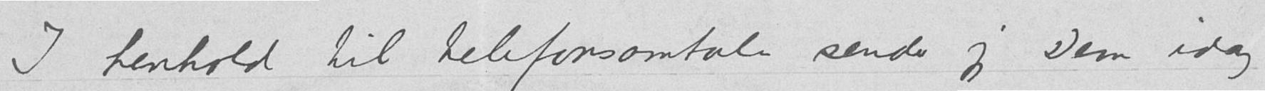I henhold til telefonsomtale sender jeg Dem idag
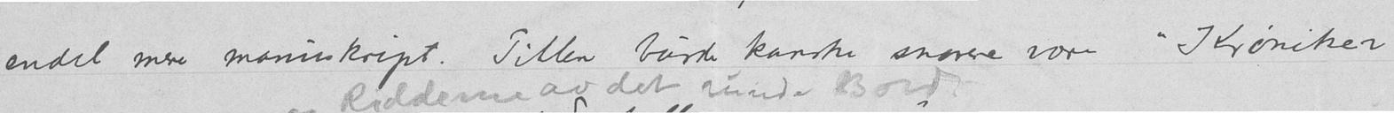endel mere manuskript. Titlen burde kanske snarere være "Krøniken
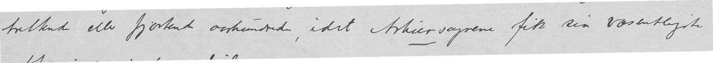trettende eller fjortende aarhundrede, idet Arturssagnene fik sin væsentligste
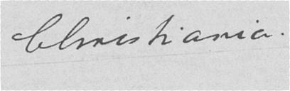Christiania.
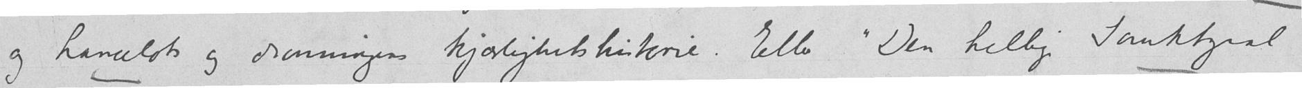og Lancelots og dronningens kjærlighetshistorie. Eller "Den hellige Sanktgral"
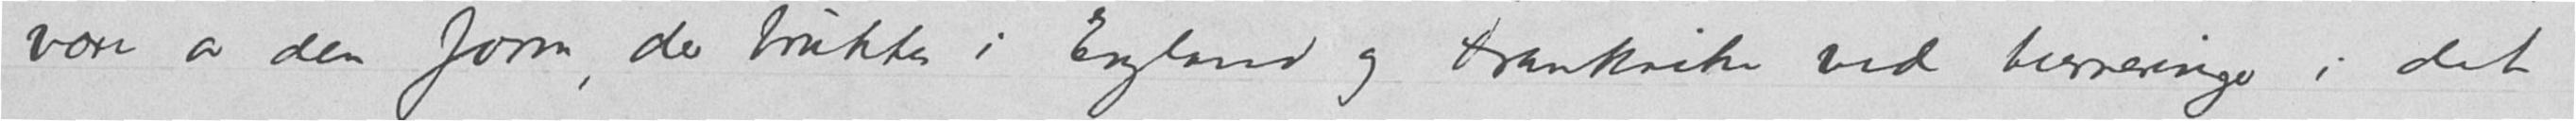være av den form, der bruktes i England og Frankrike ved turneringer i det
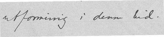utformnig i denne tid.
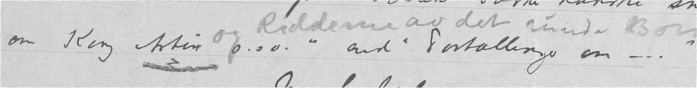om Kong Artur o.sv." end "Fortællinger om – ."
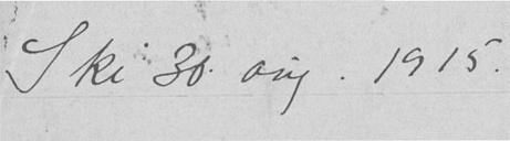Ski 30. aug. 1915.
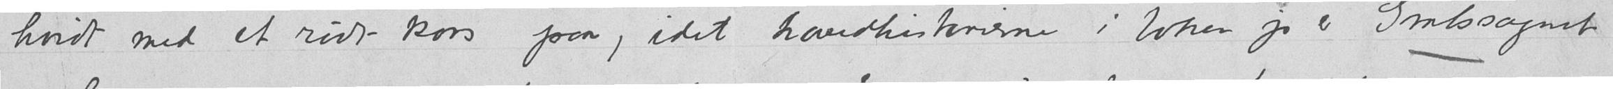hvidt med et rødt kors paa, idet hovedhistorierne i boken jo er Gralssagnet
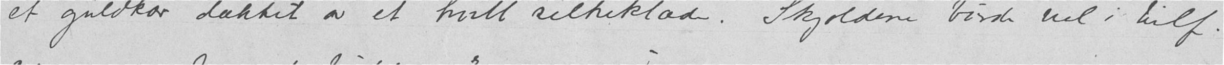et guldkar dækket av et hvitt silkeklæde. Skjoldene burde vel i tilf.
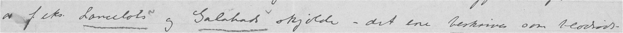av f.eks. Lancelots og Galahads skjolde – det ene beskrives som blodrødt
